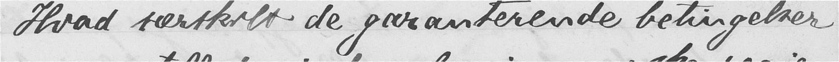Hvad særskilt de garanterende betingelser
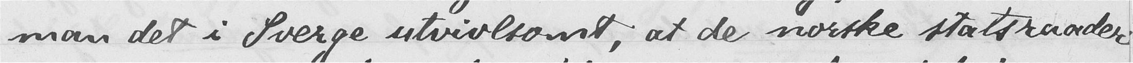man det i Sverge utvivlsomt, at de norske statsraader
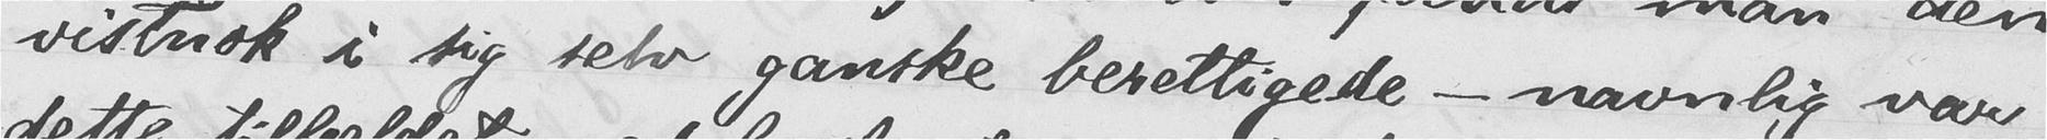vistnok i sig selv ganske berettigede - navnlig var
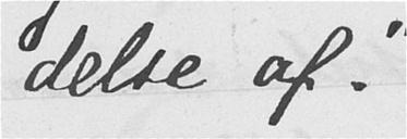delse af."
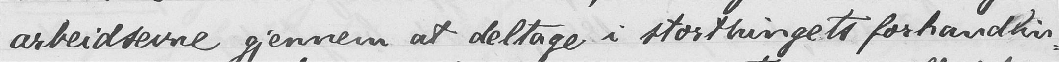arbeidsevne gjennem at deltage i storthingets forhandlin-
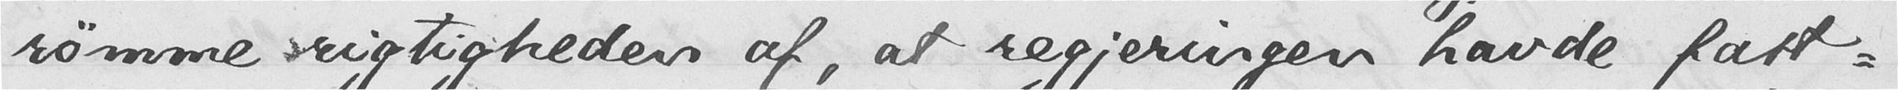rømme rigtigheden af, at regjeringen havde fast-
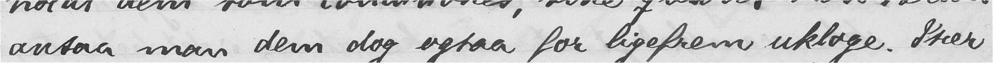ansaa man dem dog ogsaa for ligefrem ukloge. Især
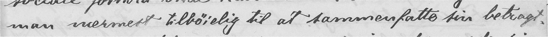man nærmest tilbøielig til at sammenfatte sin betragt-
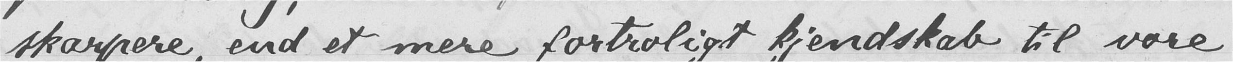skarpere, end et mere fortroligt kjendskab til vore
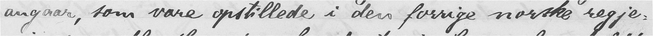angaar, som vare opstillede i den forrige norske regje-
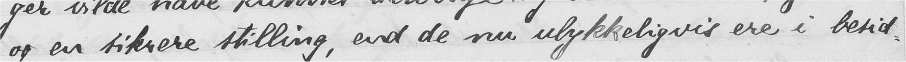og en sikrere stilling, end de nu ulykkeligvis ere i besid-
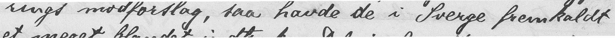rings modforslag, saa havde de i Sverge fremkaldt
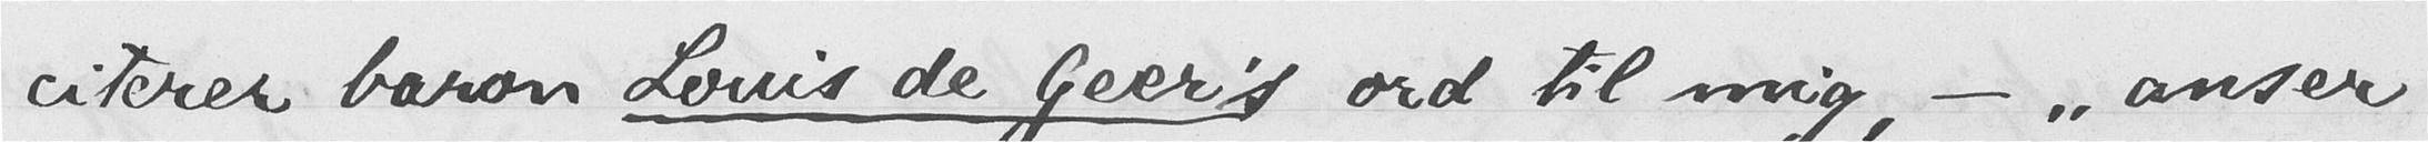citerer baron Louis de Geer's ord til mig, - "anser
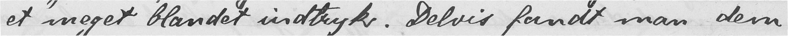et meget blandet indtryk. Delvis fandt man dem
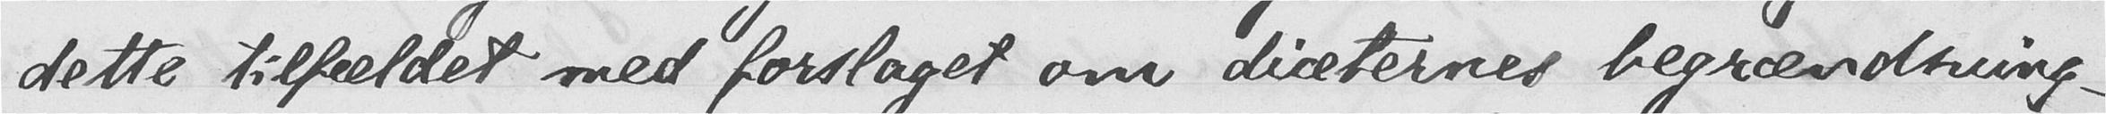dette tilfældet med forslaget om diæternes begrændsning - ;
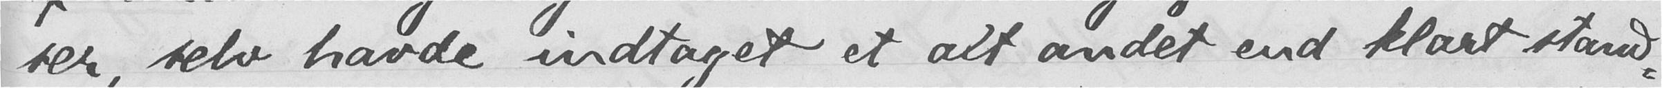ser, selv havde indtaget et alt andet end klart stand-
